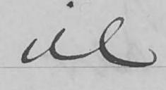vil
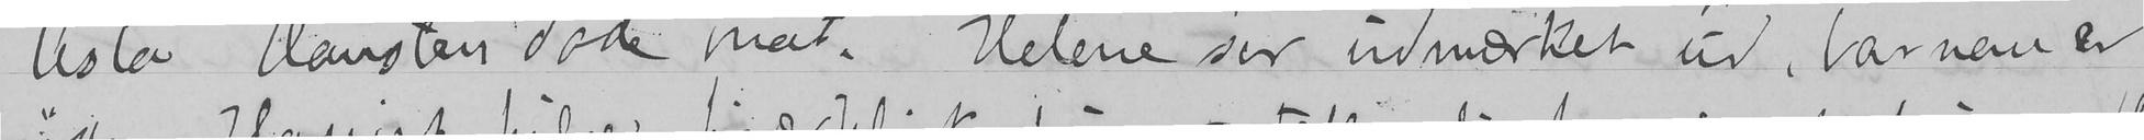Asta Hansten døde mat. Helene ser udmærket ud, barnene er
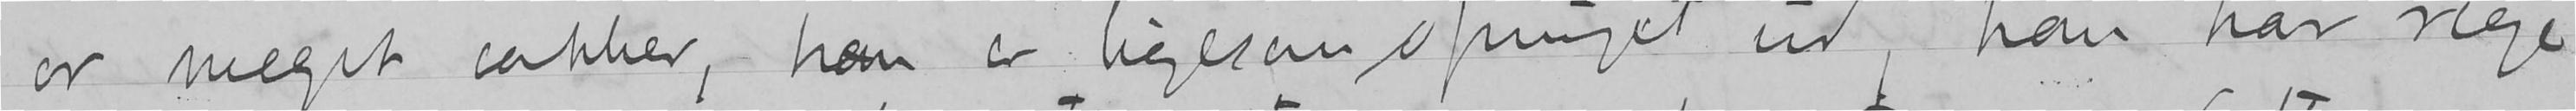er meget vakker, han er ligesom sprunget ud, han har rige
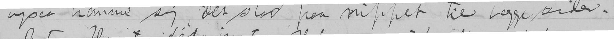ogsaa komme sig, det stod paa nippet til begge sider.
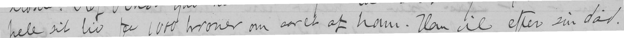hele sit liv faa 1000 kroner om aaret af ham. Han vil efter sin død
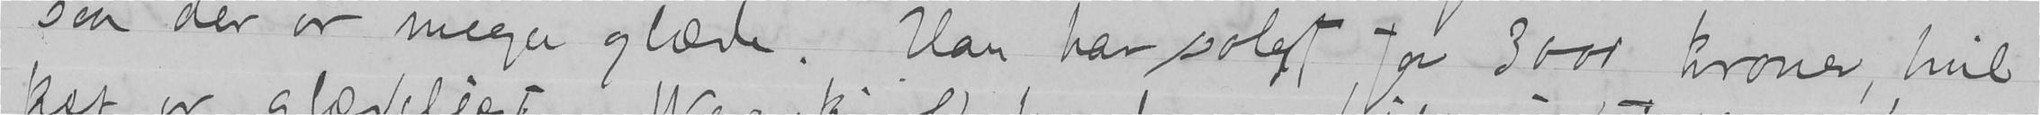saa der er megen glæde. Han har solgt for 3000 kroner, hvil-
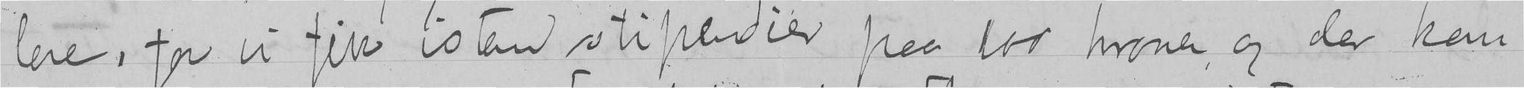lere, for vi fik istand stipendier paa 100 kroner, og der kom
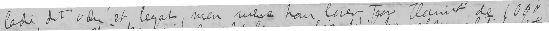lade det være et legat, men mest han lever, faar Harriet de 1000
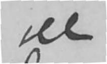vel
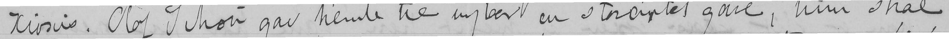Krøsus. Olaf Schou gav hende til nytaar en storartet gave, hun skal
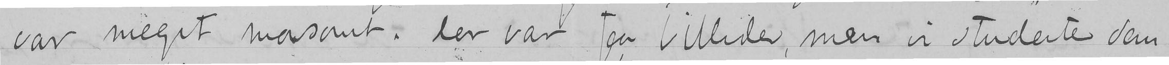var meget morsomt. Der var faa billeder, men vi studerte dem
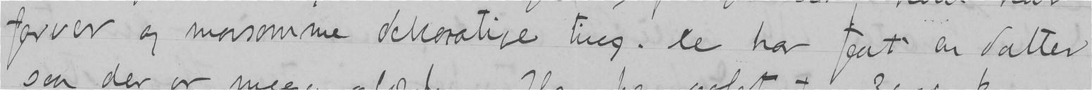farver og morsomme dekorative ting. De har faat en datter
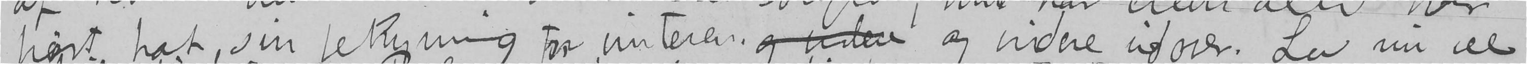høst hat sin bekymring for vinteren, og videre og videre udover. Lev nu vel
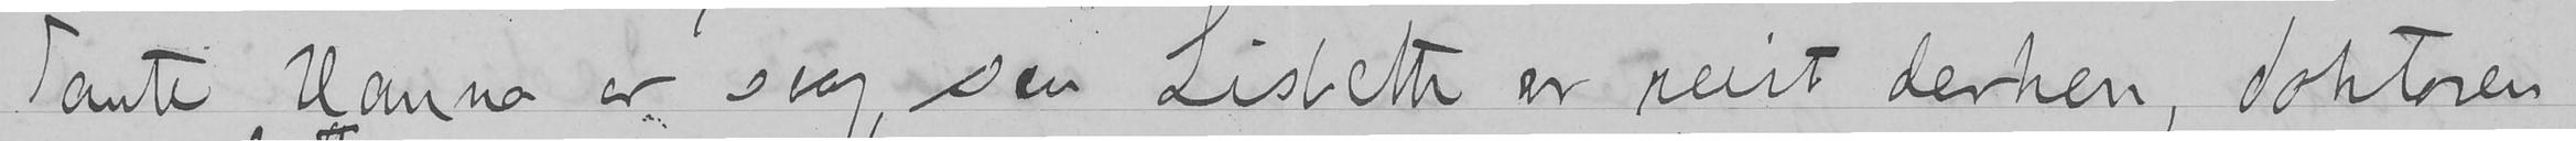Tante Hanna er svag, saa Lisbeth er reist derhen, doktoren
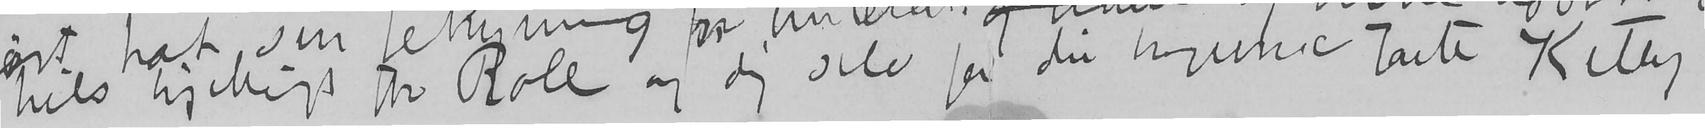hils hjerteligt fru Roll, og dig selv fra din hengivne tante Kitty
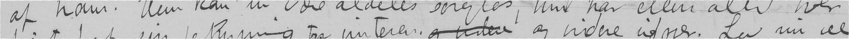af ham. Hun kan nu være aldeles sorgløs, hun har ellers altid hver
